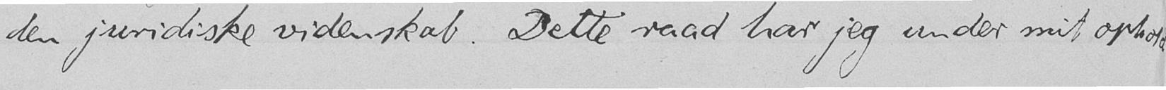den juridiske videnskab. Dette raad har jeg under mit ophold
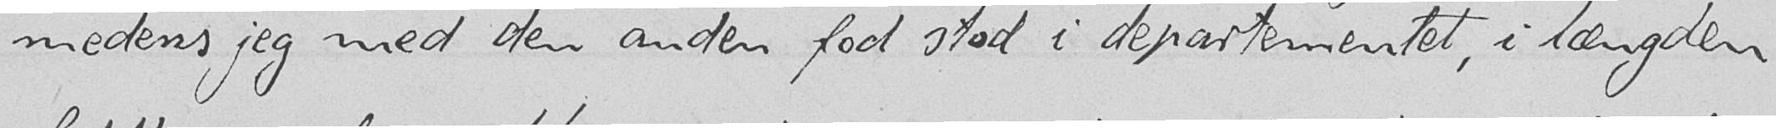medens jeg med den anden fod stod i departementet, i længden
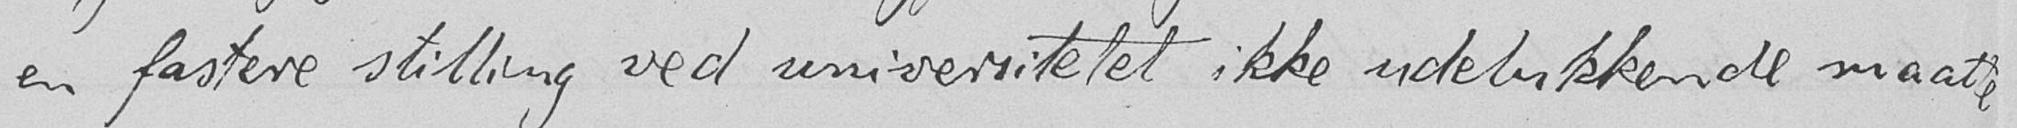en fastere stilling ved universitetet ikke udelukkende maatte
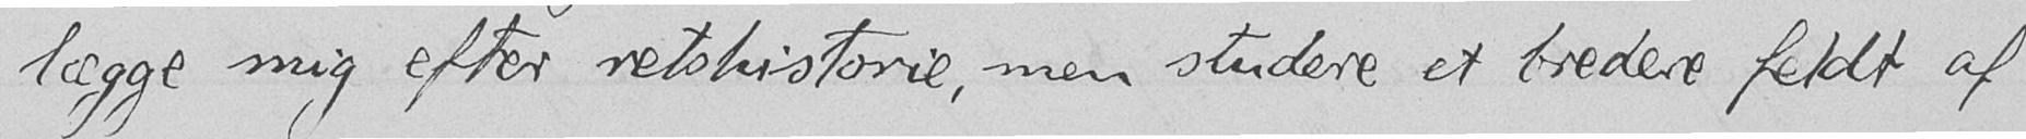lægge mig efter retshistorie, men studere et bredere feldt af
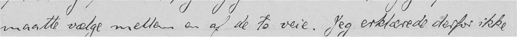maatte vælge mellem en af de to veie. Jeg erklærede derfor ikke
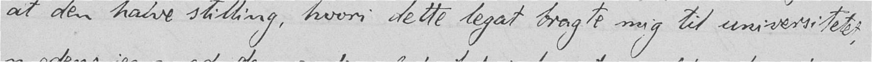at den halve stilling, hvori dette legat bragte mig til universitetet,
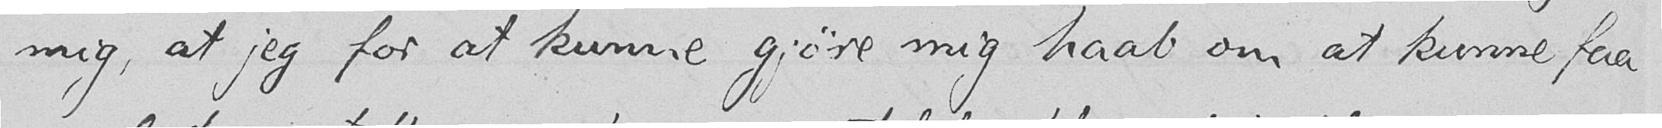mig, at jeg for at kunne gjøre mig haab om at kunne faa
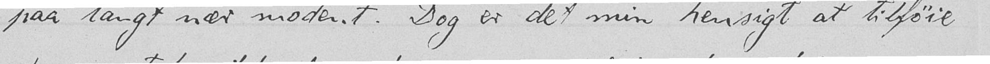paa langt nær modent. Dog er det min hensigt at tilføie
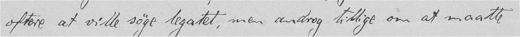oftere at ville søge legatet, men androg tillige om at maatte
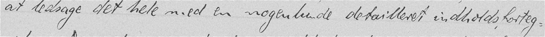at ledsage det hele med en nogenlunde detailleret indholdsforteg-
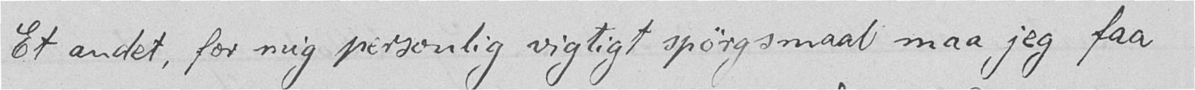Et andet, for mig personlig vigtigt spørgsmaal maa jeg faa
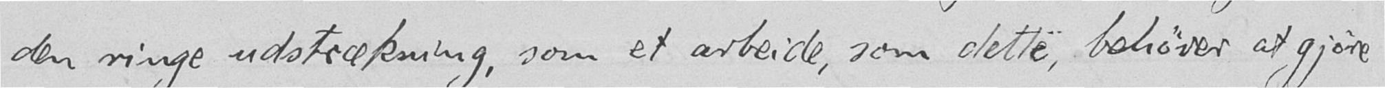den ringe udstrækning, som et arbeide, som dette, behøver at gjøre
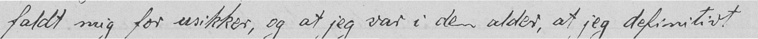faldt mig for usikker, og at jeg var i den alder, at jeg definitivt
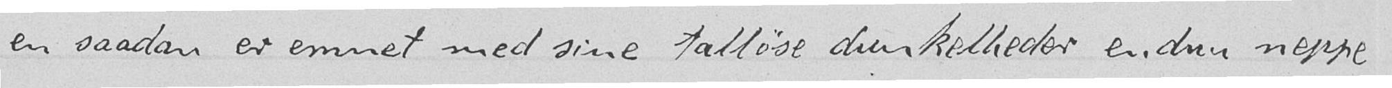en saadan er emnet med sine talløse dunkelheder endnu neppe
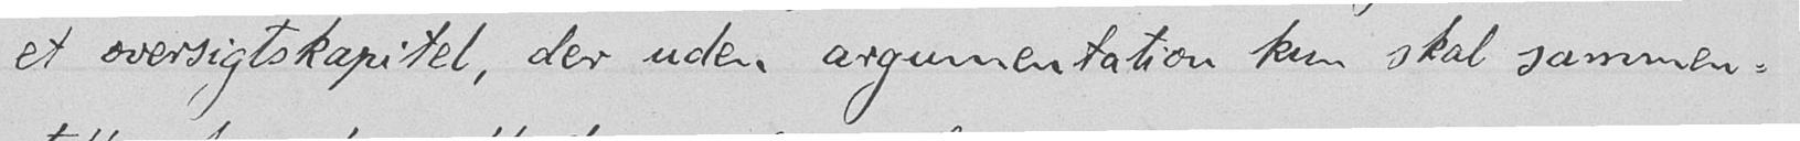et oversigtskapitel, der uden argumentation kun skal sammen-
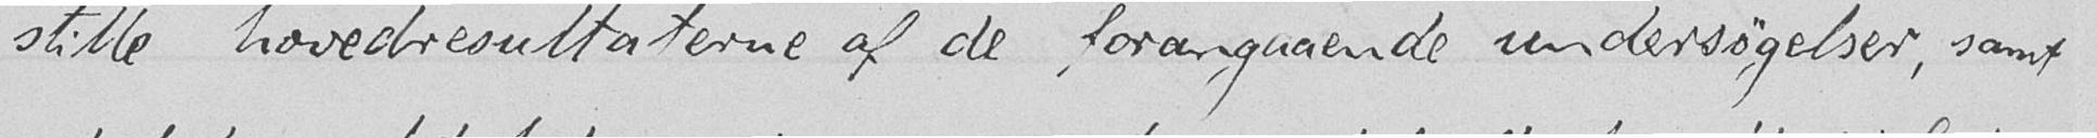stille hovedresultaterne af de forangaaende undersøgelser, samt
